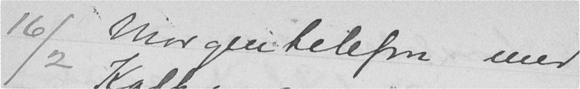16/2. Morgentelefon med
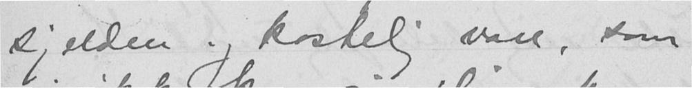sjelden og kostelig vare, som
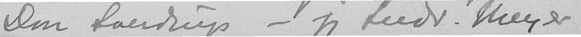Don Sverdrups - jeg tvedr. Meyer
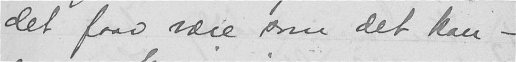det faar være som det kan -
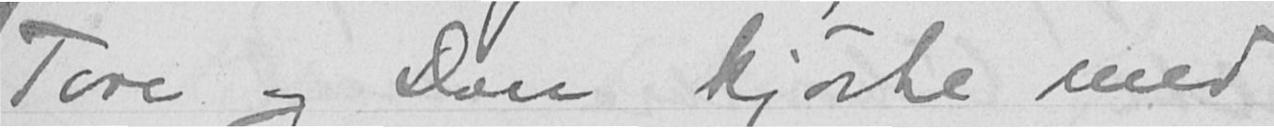Tore og Don kjørte med
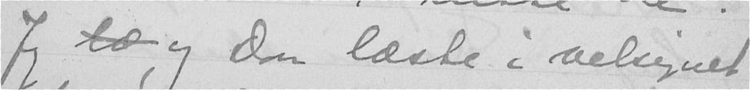Jeg læ og Don læste i velsignet
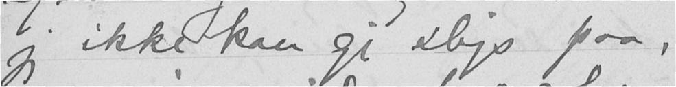jeg ikke kan gi slip paa,
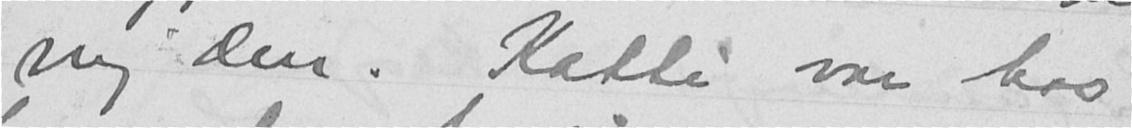mig den. Katti var hos
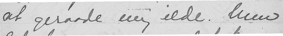at geraade mig ilde. Men
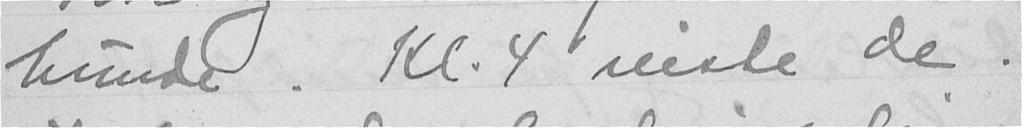hunde. Kl. 4 reiste de.
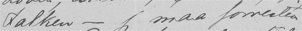Falken - jeg maa forresten
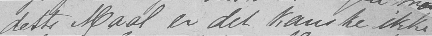dette Maal er det kanske ikke
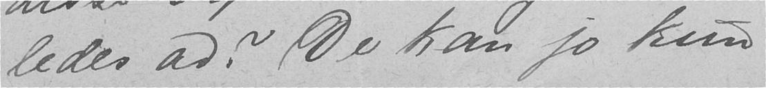ledes ad? De kan jo kun
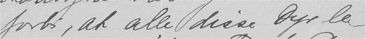forbi, at alle disse dyr le-
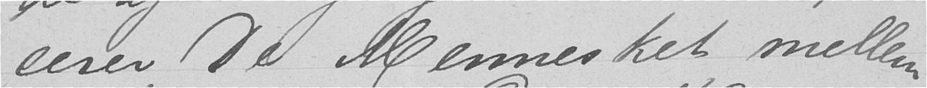cerer de Mennesket mellem
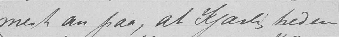mest an paa, at Kjærligheden
